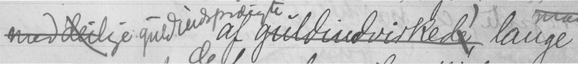guldindspridigte af lange -
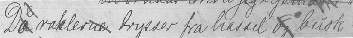Da raklerne drysser fra hassel og busk:
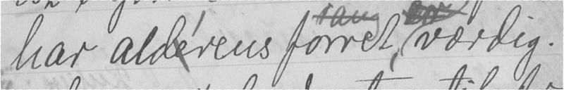- har ald'erens forret, værdig.
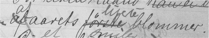af aarets hvite blommer.
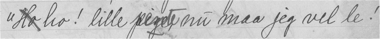"Ho ho! lille jente nu maa jeg vel le!"
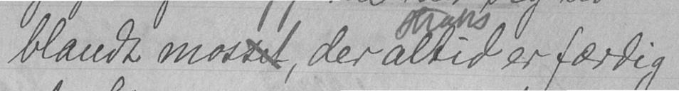blandt mosset, der straks er færdig
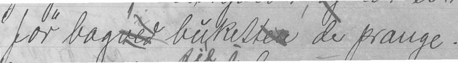før bagved buketten de prange. -
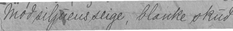Mod siljuens seige, blanke skud
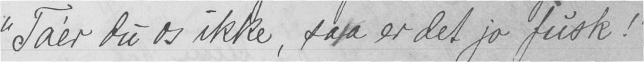"Taer du os ikke, saa er det jo fusk!"
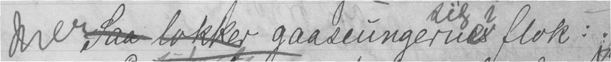men gaaseungernes sif i flok:
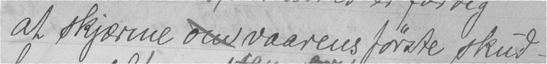at skjærme om vaarens første skud -
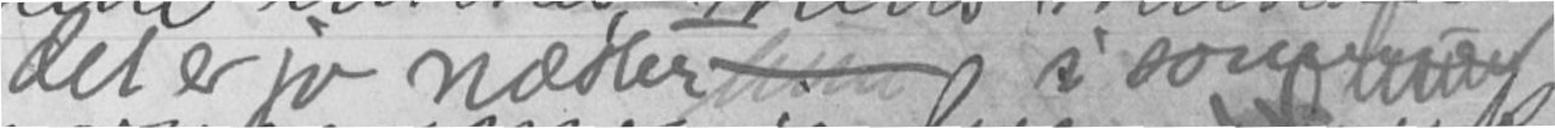det er jo næsten - i som
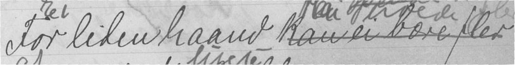For liden haand kan holde fler
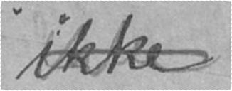ikke
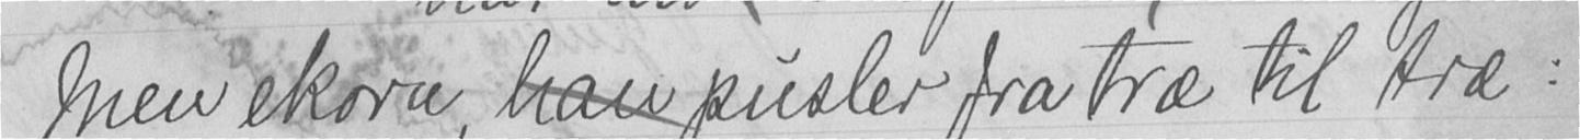Men ekorn, han pusler fra træ til træ:
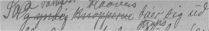Og yndig knopperne taer sig ud,
